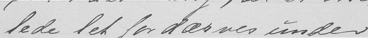lede let fordærves under
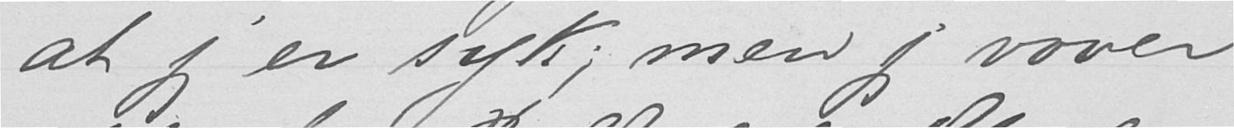at jeg er syk; men jeg vover
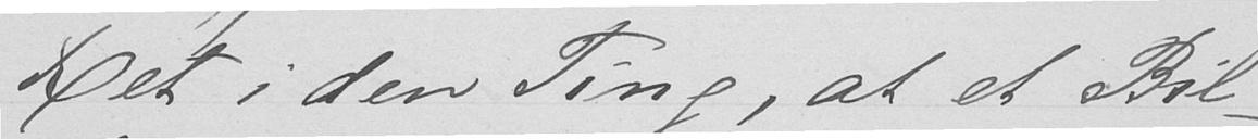Ret i den Ting, at et Bil-
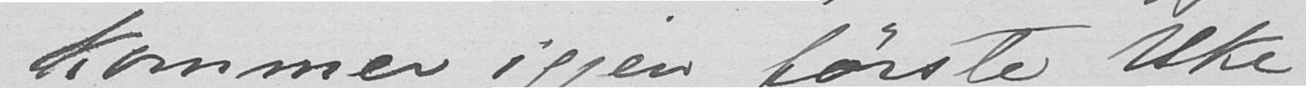kommer igjen første Uke
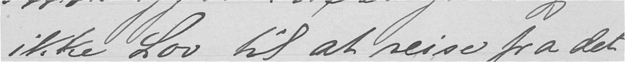ikke Lov til at reise fra det
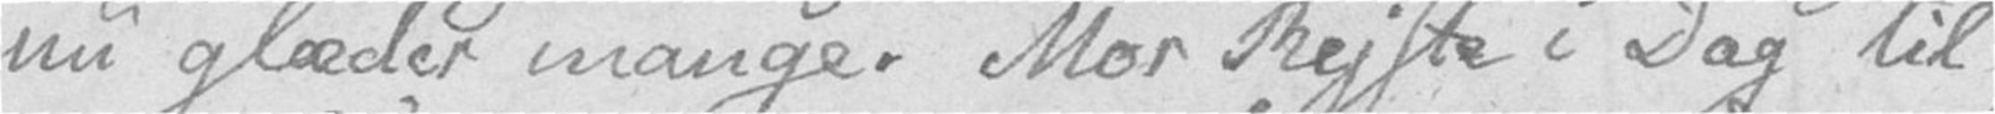nu glæder mange. Mor Rejste i Dag til
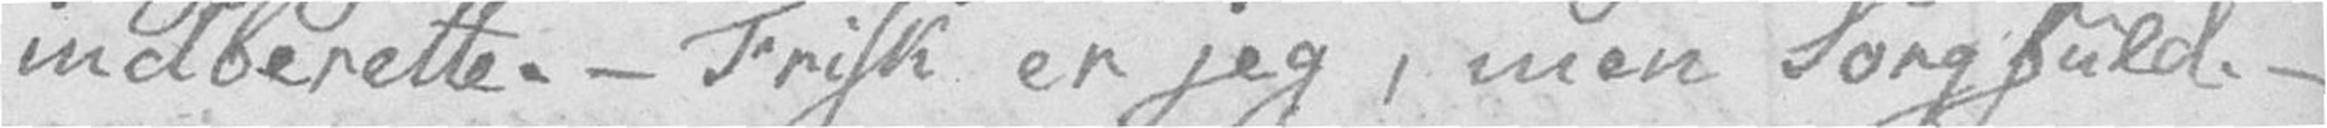indberette. – Frisk er jeg, men Sorgfuld. –
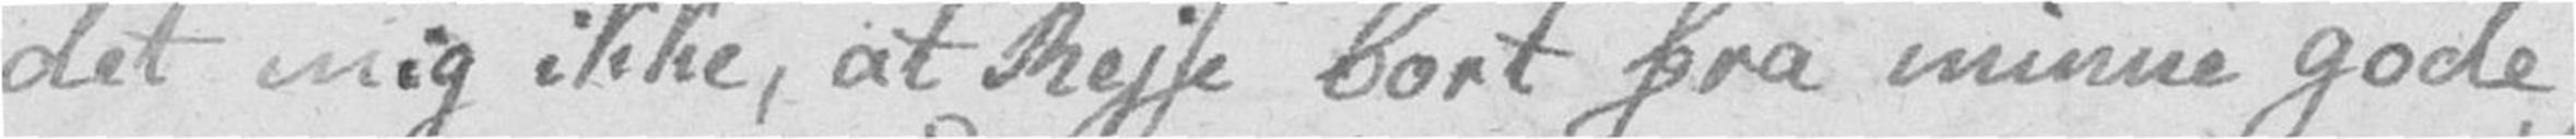det mig ikke, at Rejse bort fra minne gode
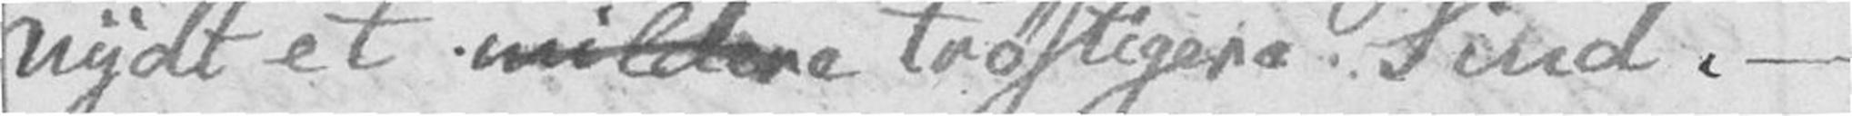nydt et mildere trøstigere Sind. –
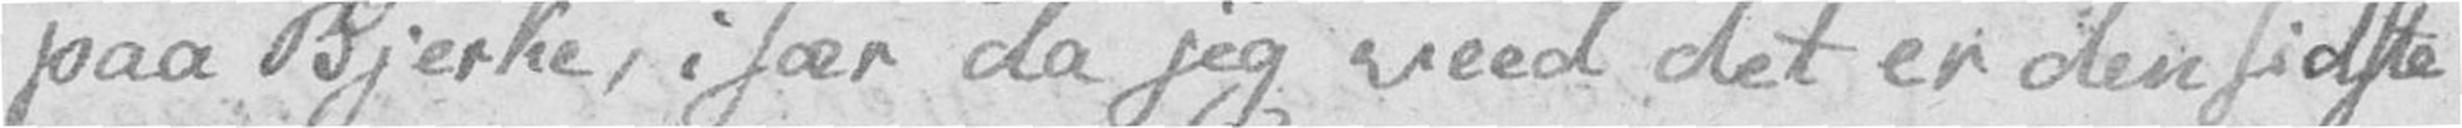paa Bjerke, især da jeg veed det er den sidste
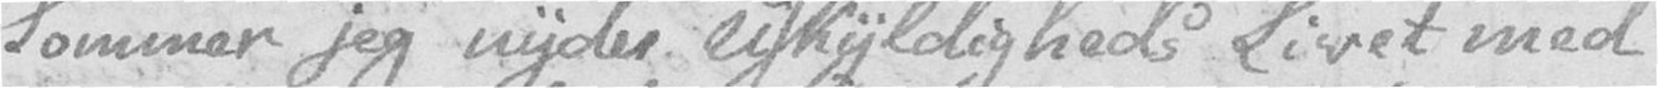Sommer jeg nyder uskyldigheds Livet med
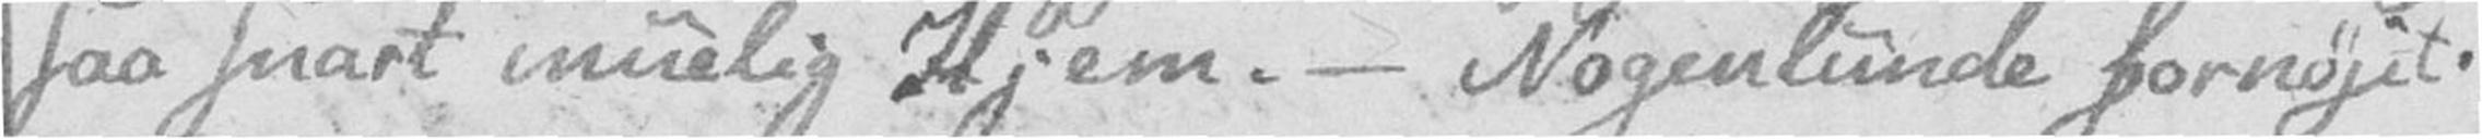saa snart muelig Hjem. – Nogenlunde fornøjet.
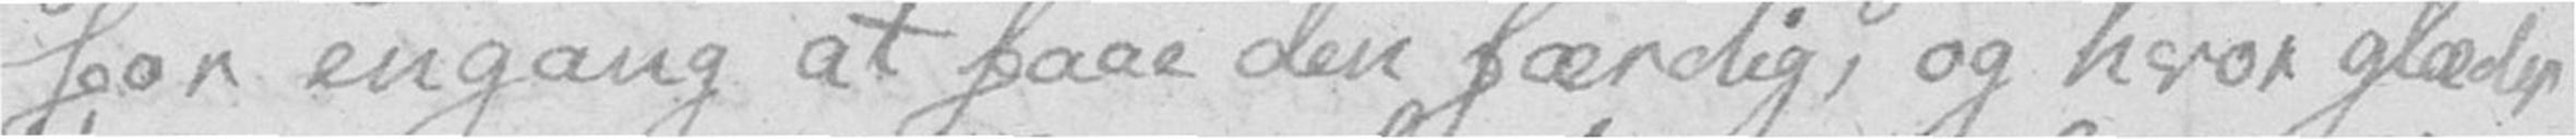for engang at faae den færdig, og hvor glæder
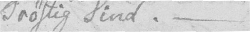Trøstig Sind. –
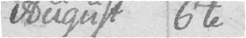August 6te
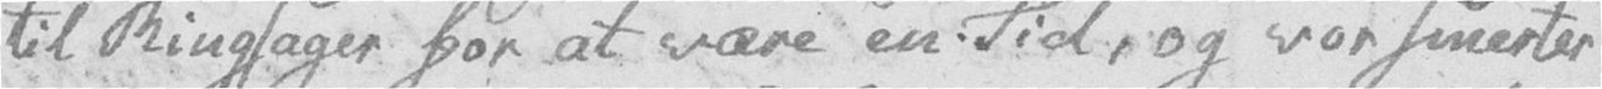til Ringsager for at være en Tid, og vor smerter
